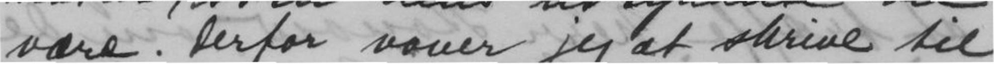være. Derfor vover jeg at skrive til
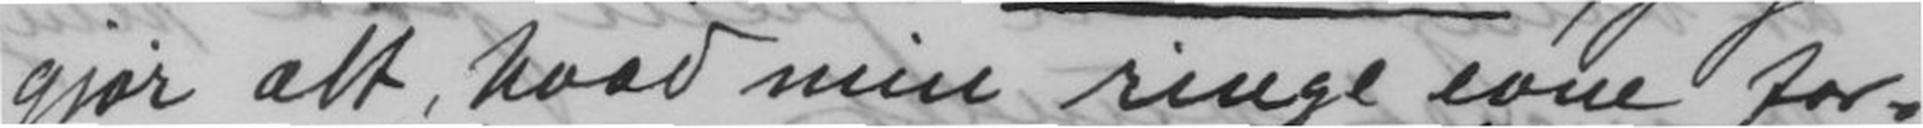gjør alt, hvad min ringe evne for-
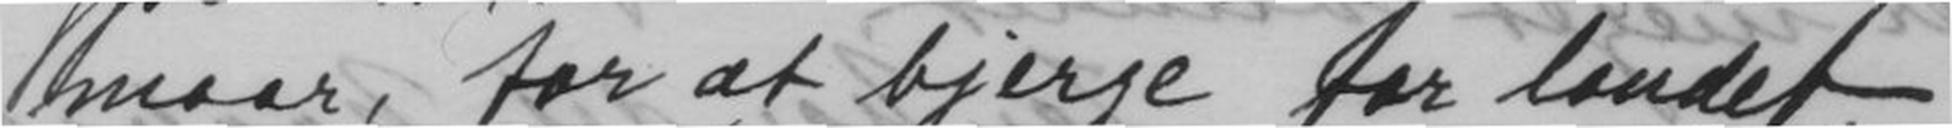maar, for at bjerge for landet.
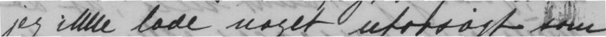jeg ikke lade noget uforsøgt som
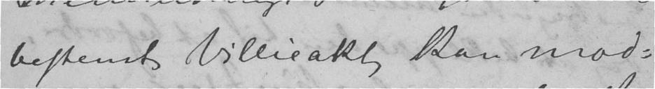bestemt Villieakt kan mod-
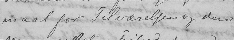maal for Tilværelsen og den
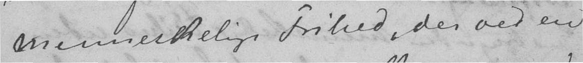menneskelige Frihed, der ved en
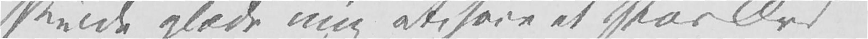skulde glæde mig at høre et Par Ord
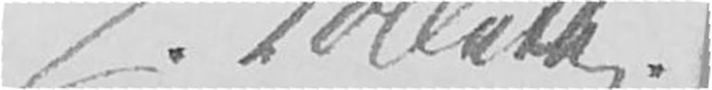C. Collett.
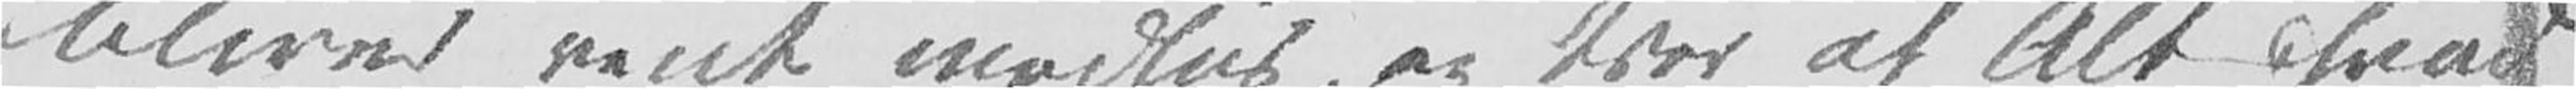bliver rent modløs, og tror at Alt hvad
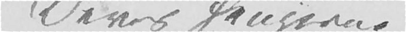Deres hengivne
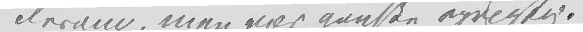derom, men vær ganske oprigtig.
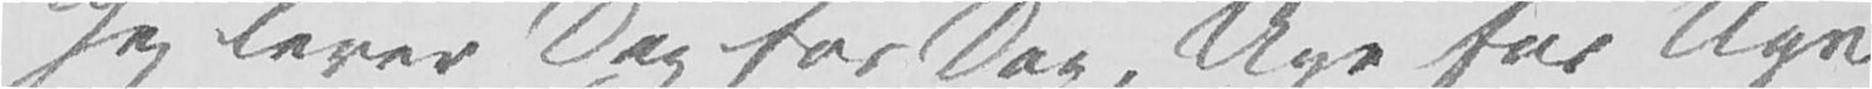Jeg lever Dag for Dag, Uge for Uge
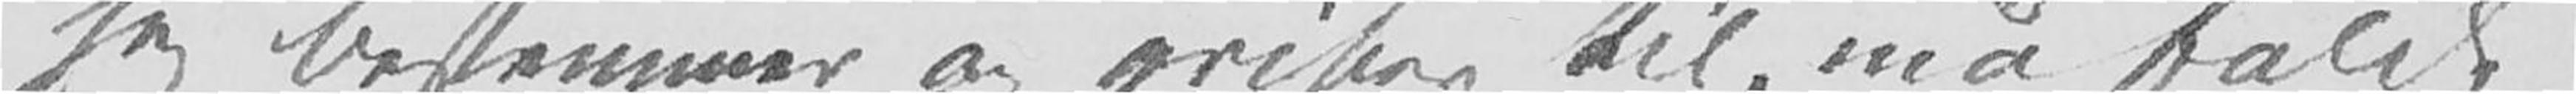jeg bestemmer og griber til, må falde
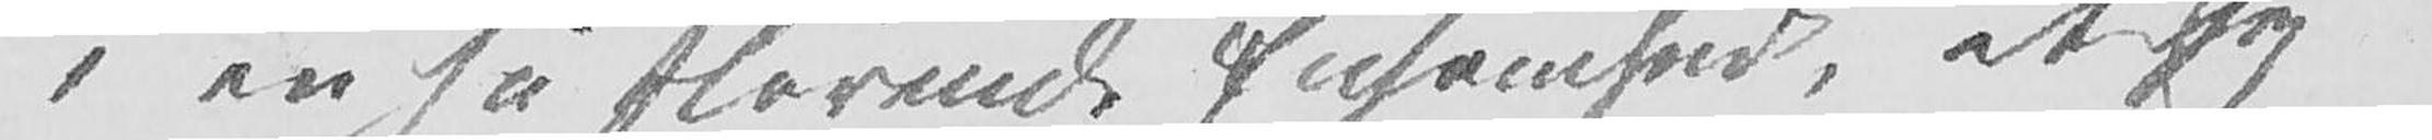i en så sløvende Ensomhed, at jeg
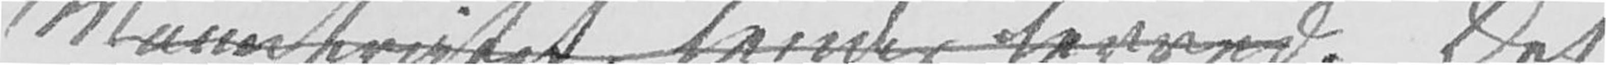Manuscriptet, sendes herved. Det
